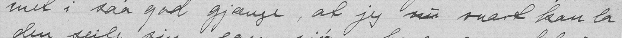met i saa god gjænge, at jeg nu saart kan la
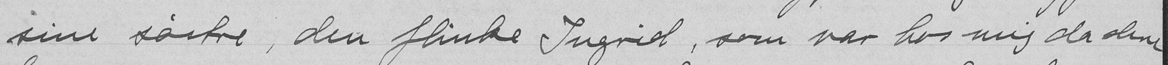sine søstre, den flinke Ingrid, som var hos mig da dere
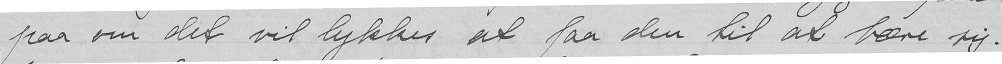paa om det vil lykkes at faa den til at bære sig.
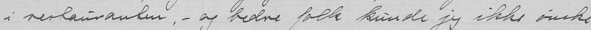i restauranten,- og bedre folk kunde jeg ikke ønske
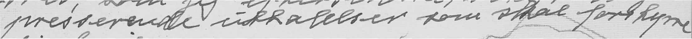presserende uttalelser som skal forstyrre
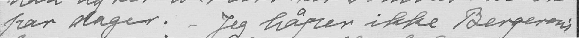par dager. - Jeg håper ikke Bergerons
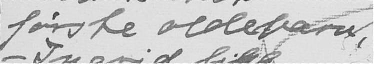første oldebarn,
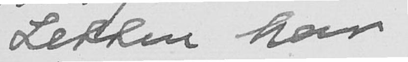Letten har
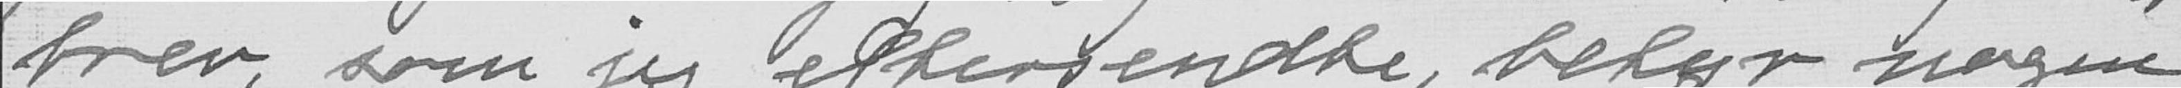brev, som jeg eftersendte, betyr nogen
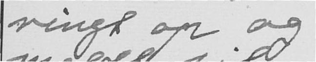ringt op og
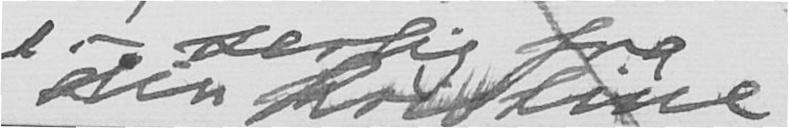din Kristine
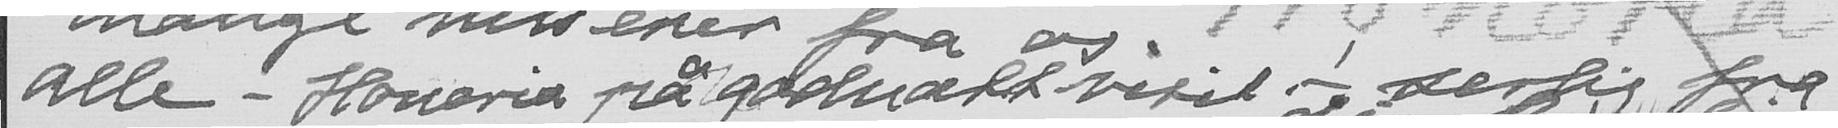alle - Honoria på godnatt visit - særlig fra
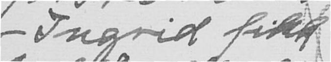- Ingrid fikk
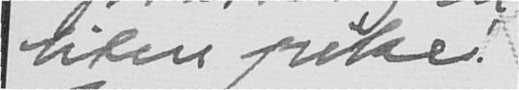liten pike.
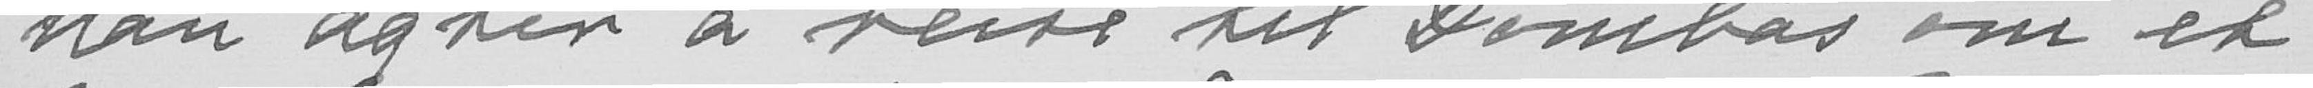han agter å reise til Dombås om et
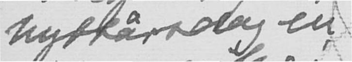nyttårsdag en
